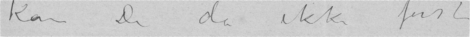kan De da ikke først
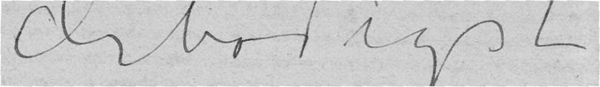Ærbødigst
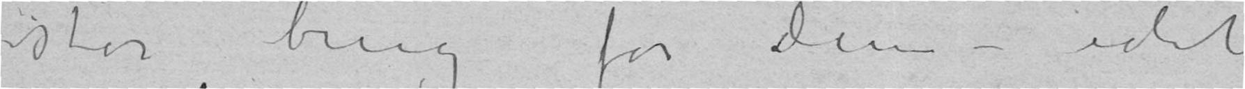stor brug for dem – idet
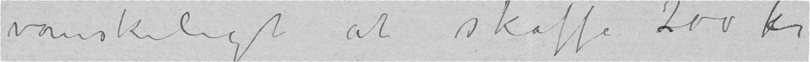vanskeligt at skaffe 200 kr.
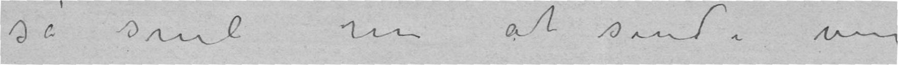så snil nu at sende mig
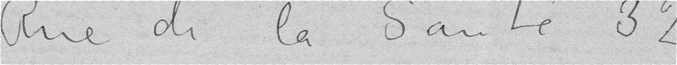Rue de la Santé 32
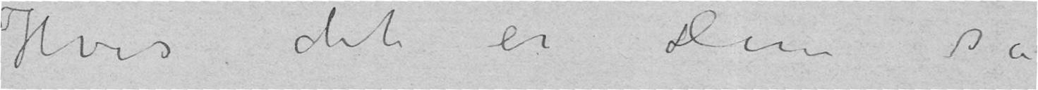Hvis det er Dem så
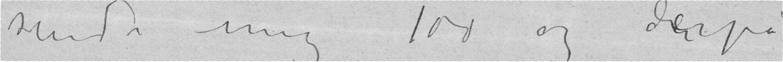sende mig 100 og derpå
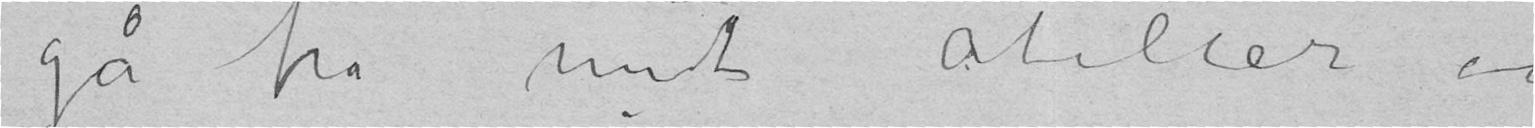gå fra mit atelier og
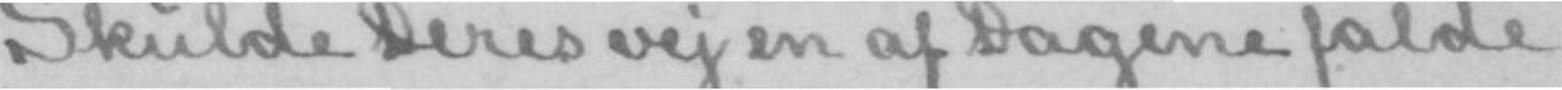Skulde Deres vej en af Dagene falde
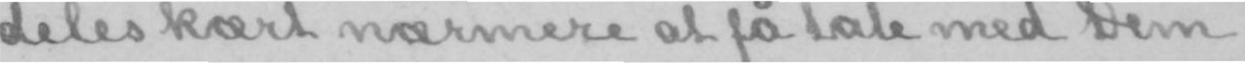deles kært nærmere at få tale med Dem
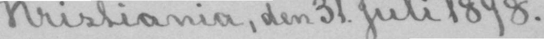Kristiania, den 31. Juli 1898.
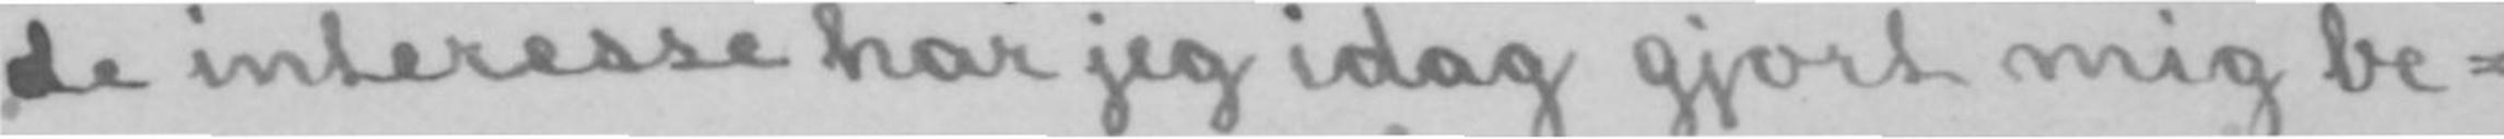de interesse har jeg idag gjort mig be-
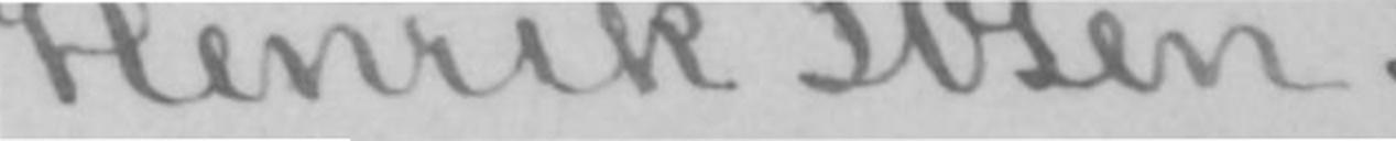Henrik Ibsen.
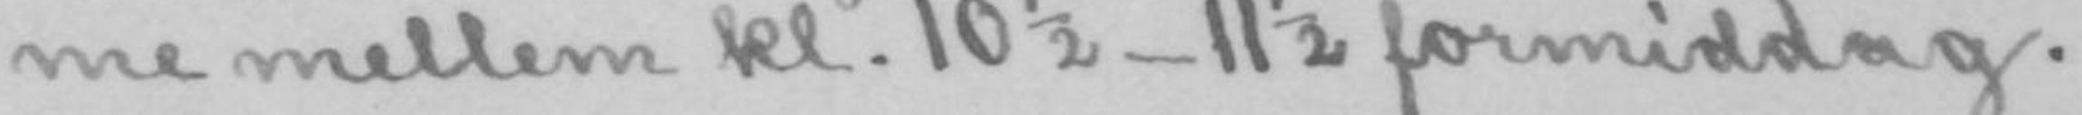me mellem kl. 10½-11½ formiddag.
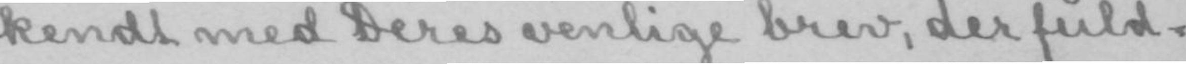kendt med Deres venlige brev, der fuld-
